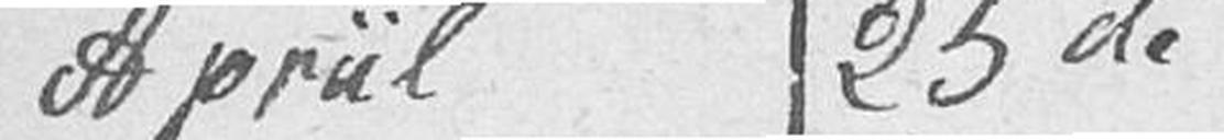Apriil 25de
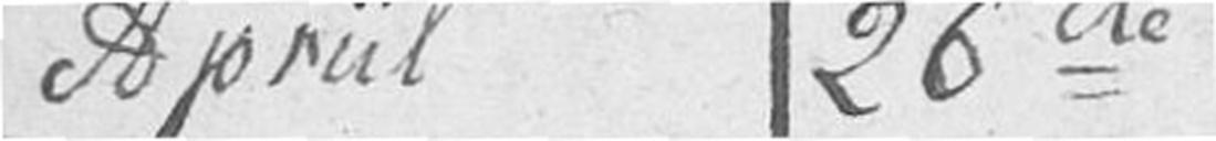Apriil 26de
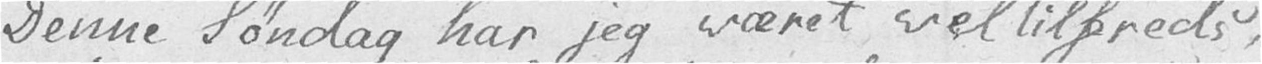Denne Søndag har jeg været veltilfreds,
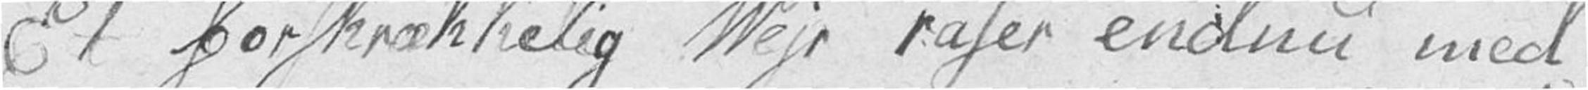Et forskrækkelig Vejr raser endnu med
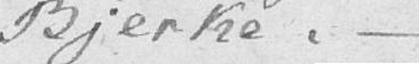Bjerke. –
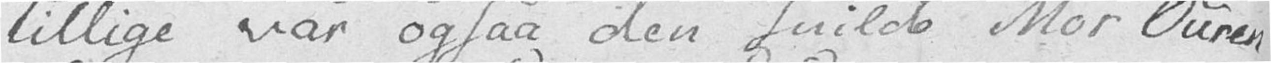tillige var ogsaa den snilde Mor Ouren
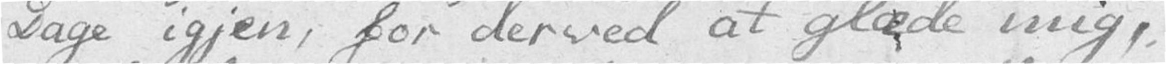Dage igjen, for derved at glæde mig,
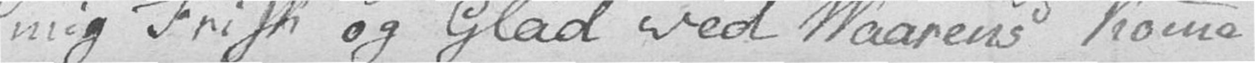mig Frisk og Glad ved Vaarens komme
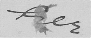her
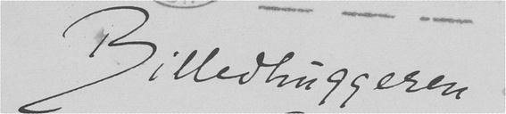Billedhuggeren
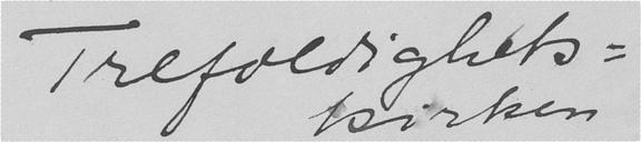Trefoldighets=
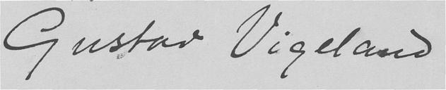Gustav Vigeland
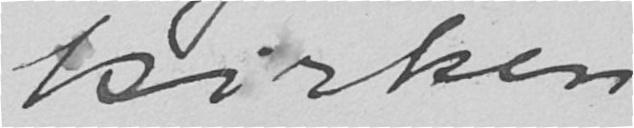kirken
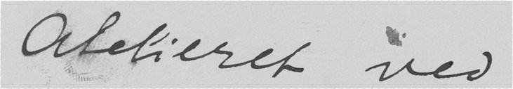Atelieret ved
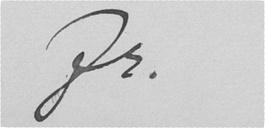fr.
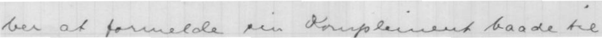ber at formelde sin Kompliment baade til
